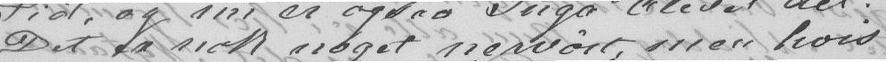Det er nok noget nervøst, men hvis
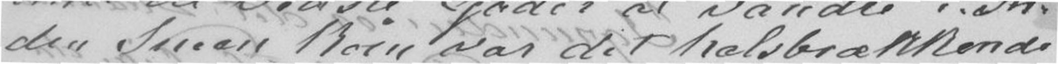den Sneen kom var det halsbrækkende
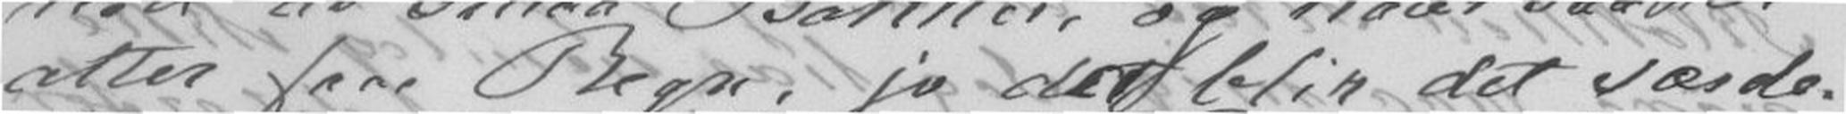atter faar Regn, jo det blir det særde-
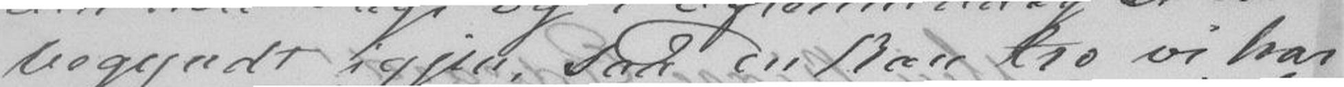begyndt igjen, saa Du kan tro vi har
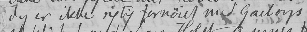Jeg er ikke rigtig fornøiet med Garborgs
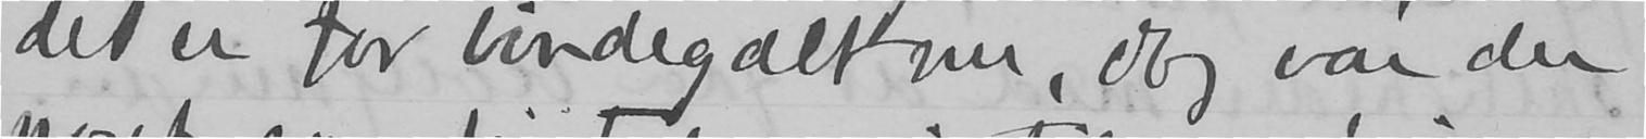det er for bindegalt nu, dog var der
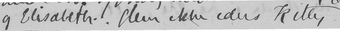og Elisabeth. Glem ikke eders Kitty.
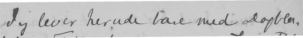Jeg lever herude bare med Dagbla-
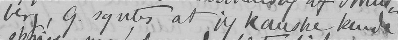berg, G. syntes at jeg kanske kunde
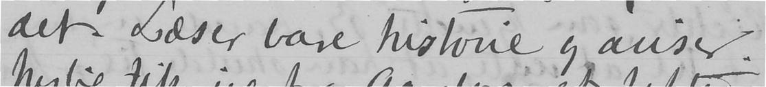det. Læser bare historie og aviser.
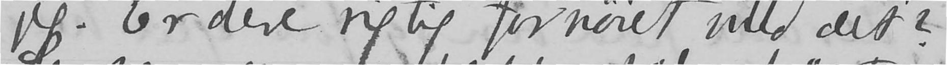jeg. Er dere rigtig fornøiet med det?
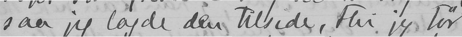saa jeg lagde den tilside, thi jeg tør
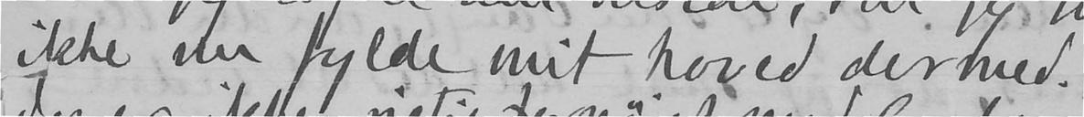ikke nu fylde mit hoved dermed.
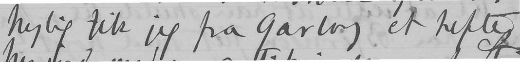Nylig fik jeg fra Garborg et hefte
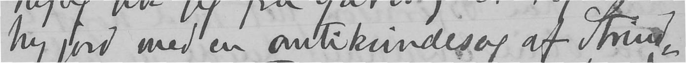Ny jord med en antikvindesag af Strind-
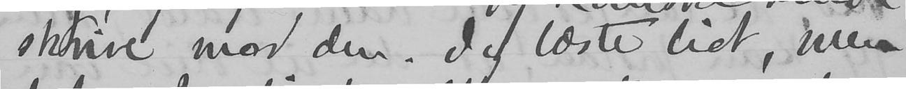skrive mod den. Jeg læste lidt, men
